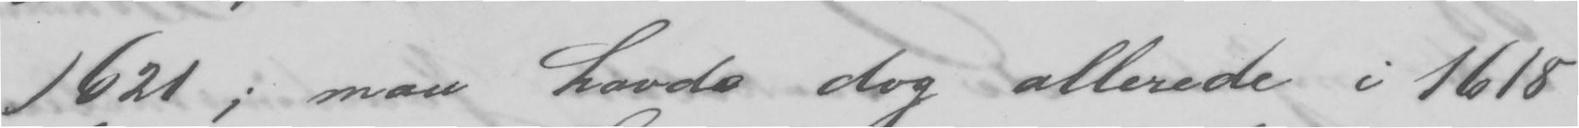1621; man havde dog allerede i 1618
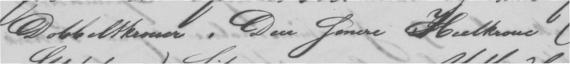Dobbeltkroner. Den senere Helkrone (
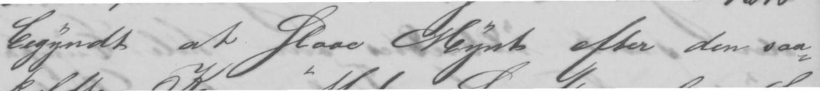begyndt at Slaae Mynt efter den saa-
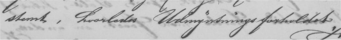stemt, hvorledes Udmyntningsforholdet
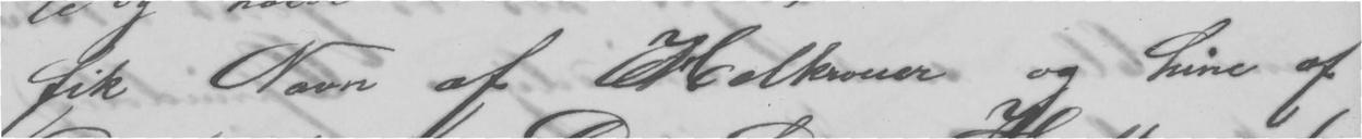fik Navn af Helkroner og hine af
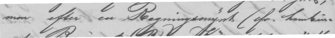men efter en Regningsmynt (fr. hambur-
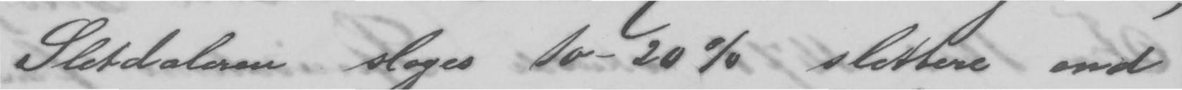Sletdaleren sloges 10-20% slettere end
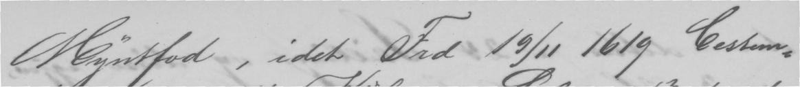Myntfod, idet Frd 19/11 1619 bestem-
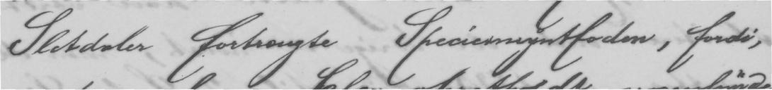Sletdaler fortrængte Speciesmyntfoden, fordi,
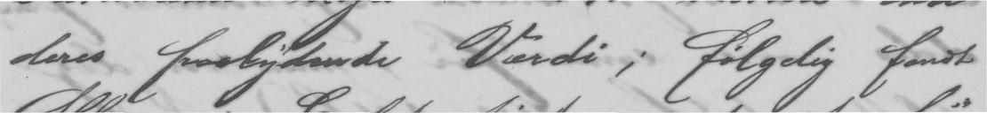deres paalydende Værdi; følgelig fandt
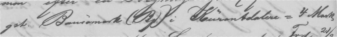g st. Bancomark (By) i Kurantdalere = 4 Mark
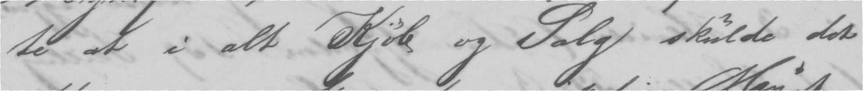te at i alt Kjøb og Salg skulde det
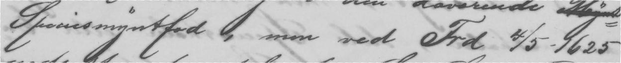Speciesmyntfod, men ved Frd 4/5-1625
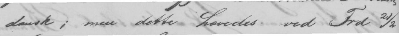dansk; men dette hævedes ved Frd 21/2
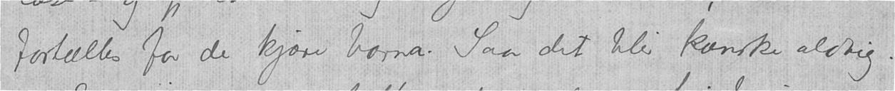fortælles for de kjære barna. Saa det blir kanske aldrig.
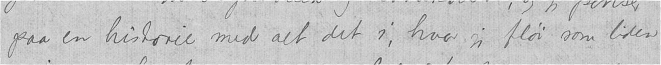paa en historie med alt det i, hvor jeg fløi som liden
